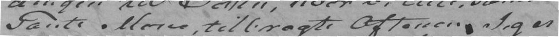Tante Mone, tilbragte Aftenen. Jeg er
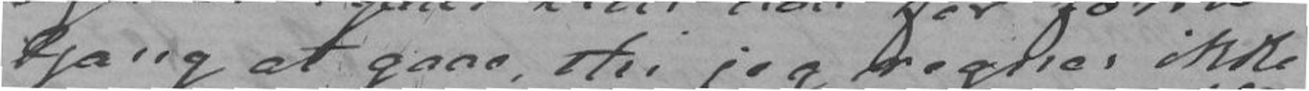Gang at gaae, thi jeg regnes ikke
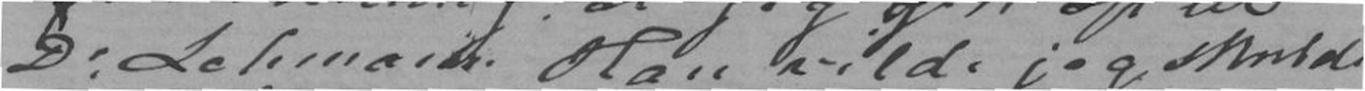Dr Lehmann. Han vilde jeg skulde
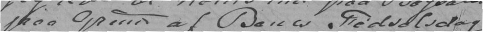paa Grund af Benes Fødselsdag,
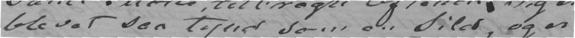blevet saa tynd som en Sild, og er
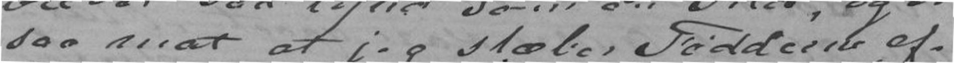saa mat at jeg slæber Fødderne ef-
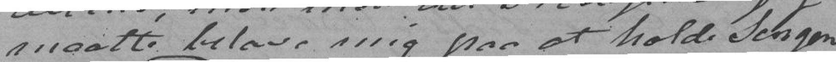maatte belave mig paa at holde Sengen
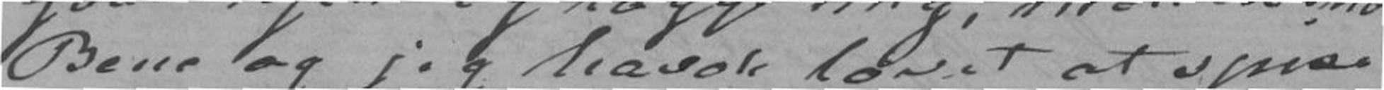Bene og jeg havde lovet at spise
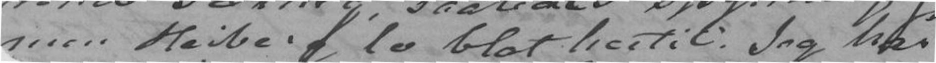men Heiberg lo blot hertil. Jeg har
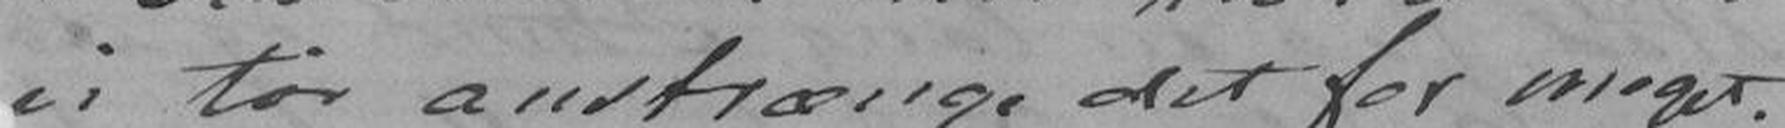ei tør anstrænge det for meget.
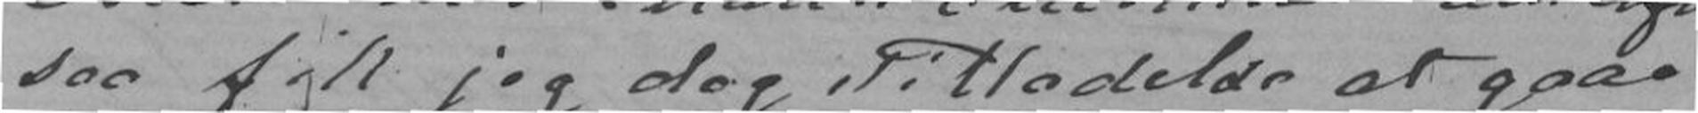saa fik jeg dog Tilladelse at gaae
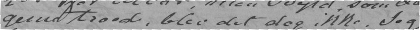gerne troede, blev det dog ikke. Jeg
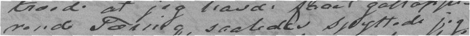rende Tæring, saaledes spyttede jeg
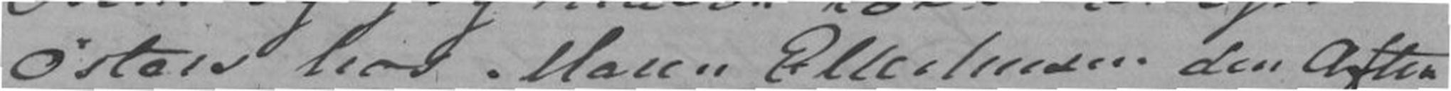Østers hos Maren Ellerhusen den Aften,
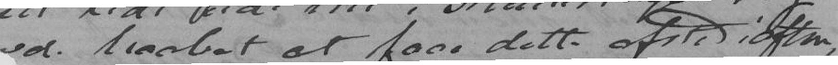vd haabet at faae dette afsted i aften,
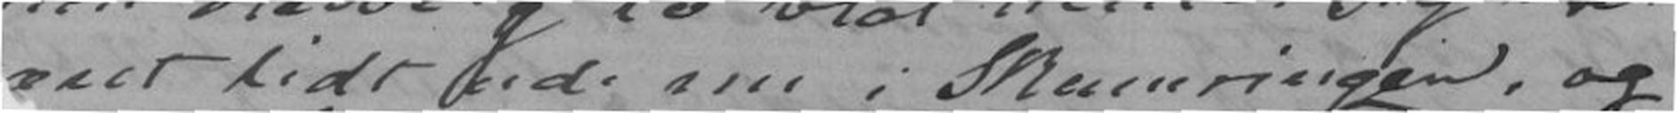æret lidt ud nu i Skumringen, og
